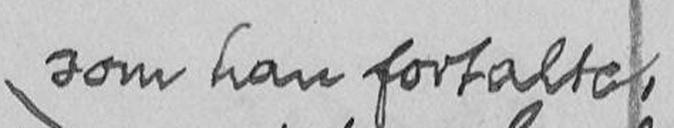som han fortalte,
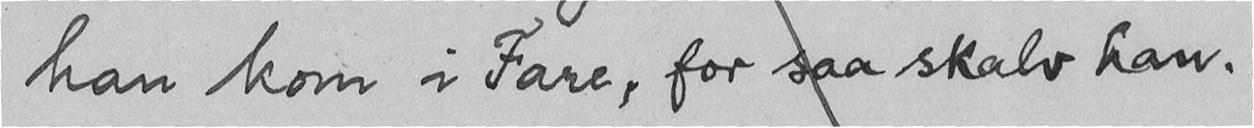han kom i Fare, for saa skalv han.
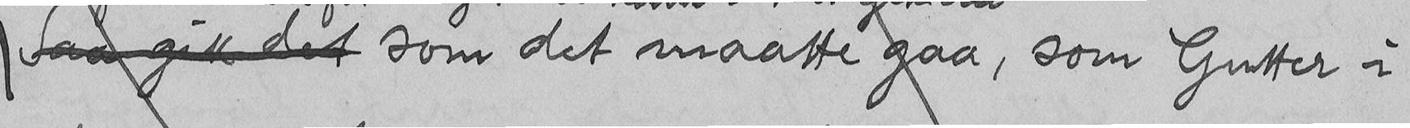Saa gik det som det maatte gaa, som Gutter i
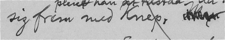sig frem med Knep, Men
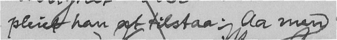pleiet han at tilstaa. Aa men
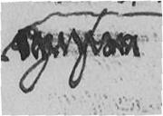synfan
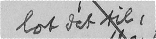lot det til,
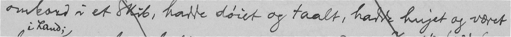ombord i et Skib, hadde døiet og taalt, hadde hujet og været
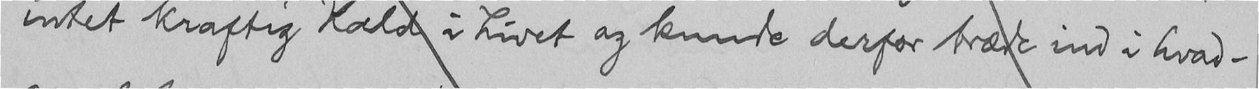intet kraftig Kald i Livet og kunde derfor træde ind i hvad-
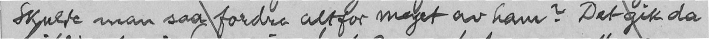Skulde man saa fordre altfor meget av ham? Det gik da
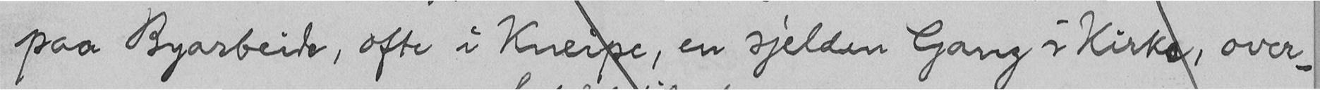paa Byarbeide, ofte i Kneipe, en sjelden Gang i Kirke, over-
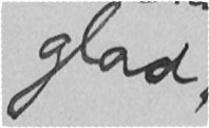glad,
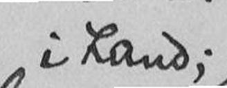i Land;
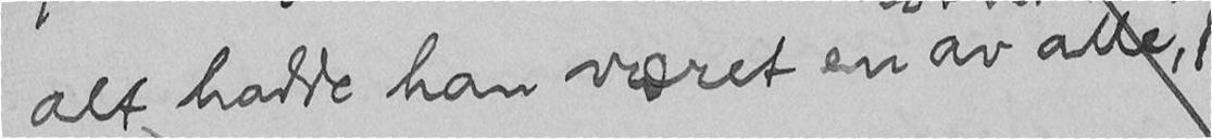alt hadde han været en av alle,
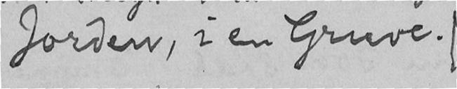Jorden, i en Gruve.
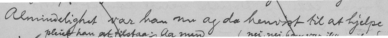Almindelighet var han nu og da henvist til at hjelpe
